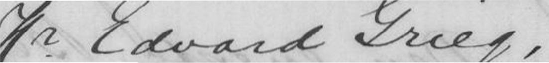Hr Edvard Grieg,
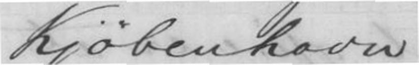Kjøbenhavn
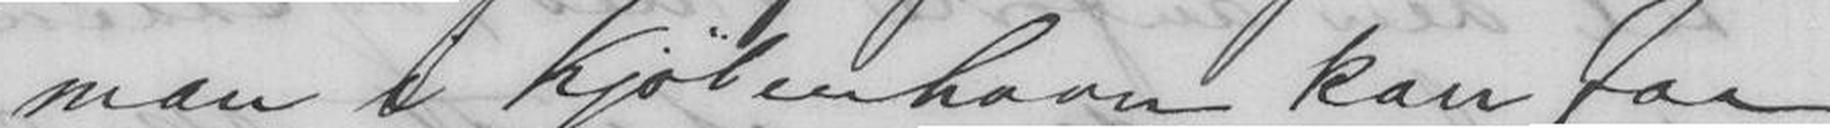man i Kjøbenhavn kan faa
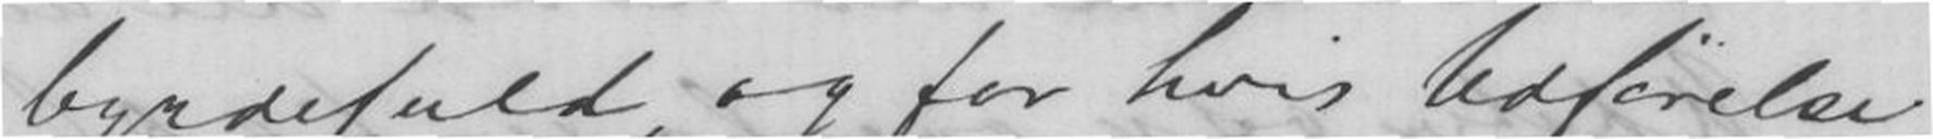byrdefuld, og for hvis Udførelse
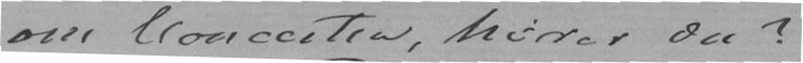om Concerten, hører du?
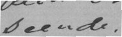seende.
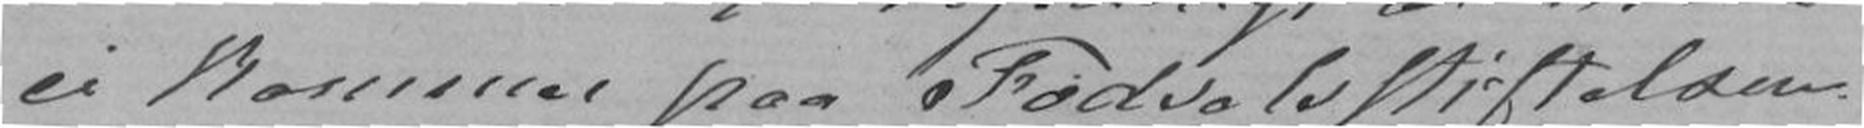ei kommer paa Fødselsstiftelsen.
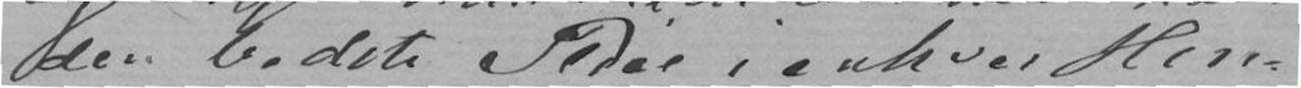den bedste Pleie i enhver Hen-
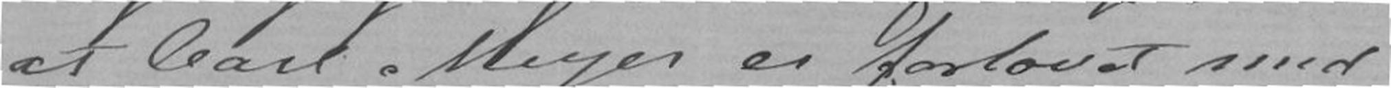at Carl Meyer er forlovet med
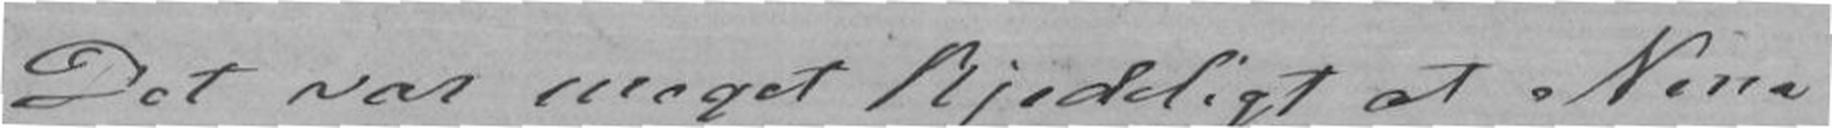Det var meget kjedeligt at Nina
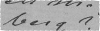berg?
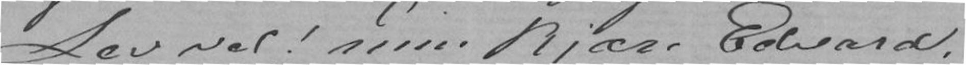Lev vel! min kjære Edvard,
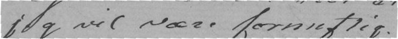jeg vil være fornuftig.
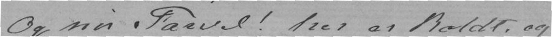Og nu Farvel! her er koldt, og
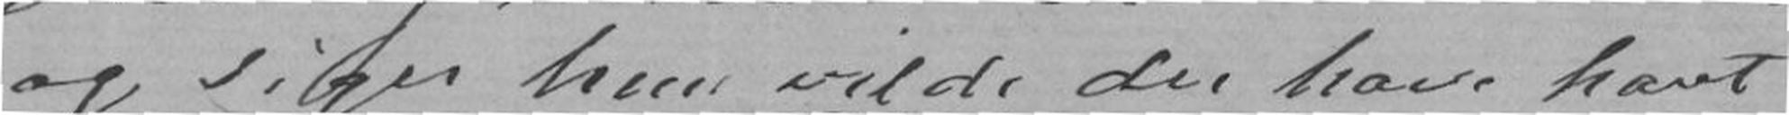og siger hun vilde der have havt
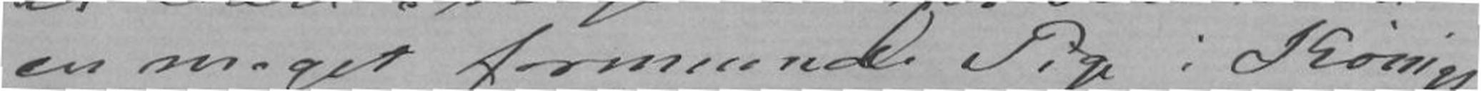en meget Fremende Pige i Kønigs-
